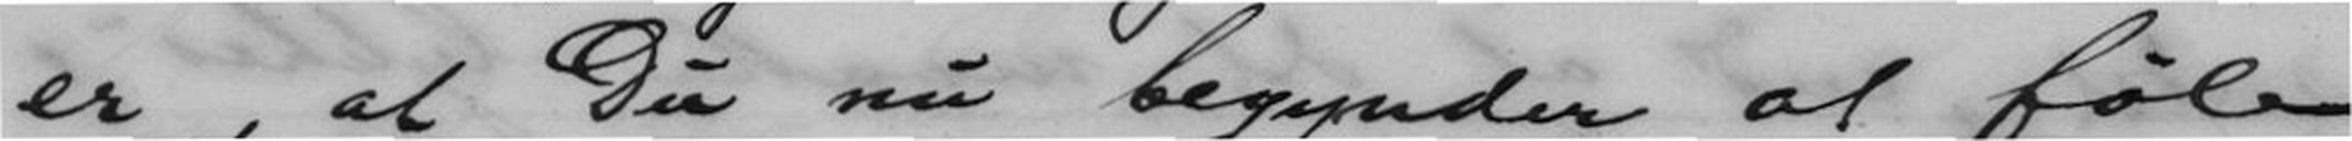er, at Du nu begynder at føle
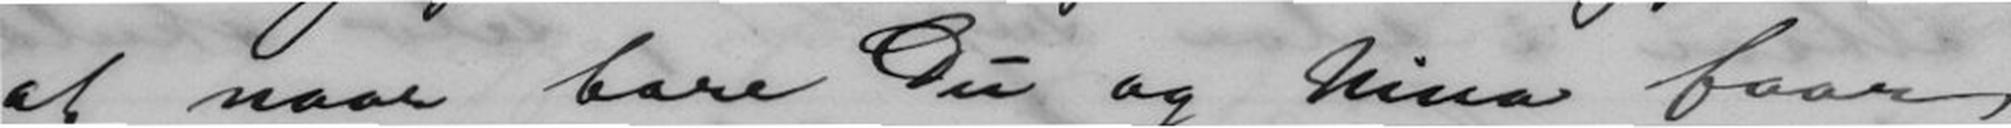at naar bare Du og Nina faar
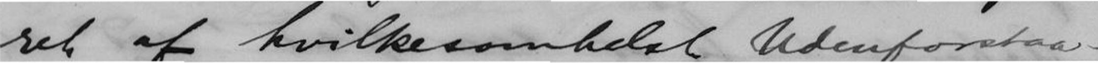ret af hvilkesomhelst Udenforstaa-
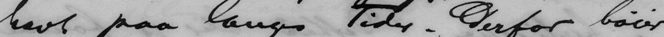havt paa lange Tider. Derfor bøier
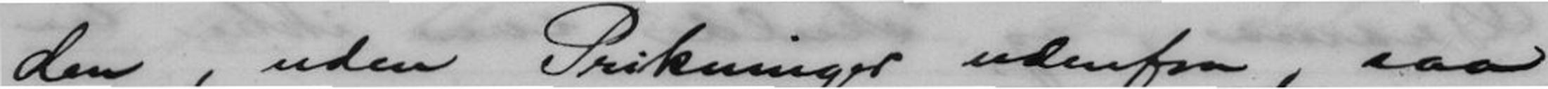den, uden Prikninger udenfra, saa
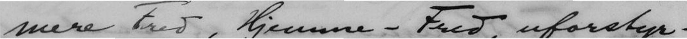mere Fred, Hjemme-Fred, uforstyr-
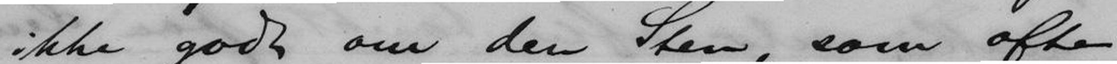ikke godt om den Sten, som ofte
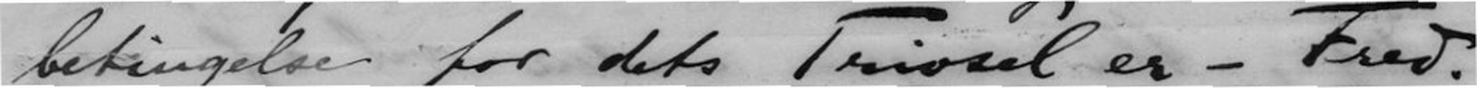betingelse for dets Trivsel er - Fred.
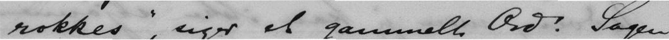rokkes", siger et gammelt Ord. Sagen
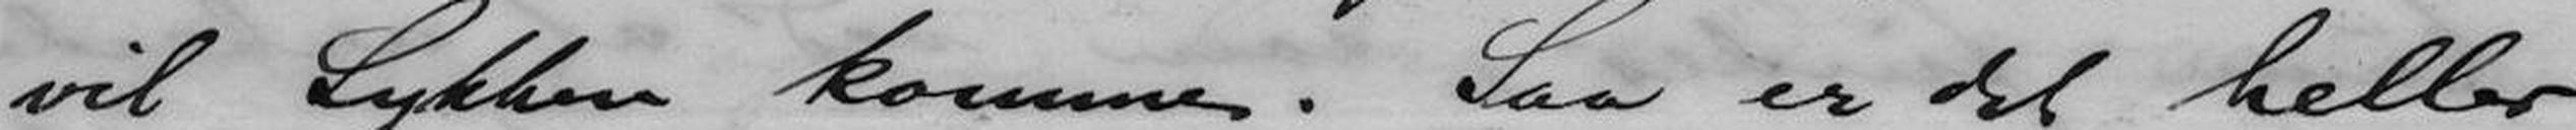vil Lykken komme. Saa er det heller
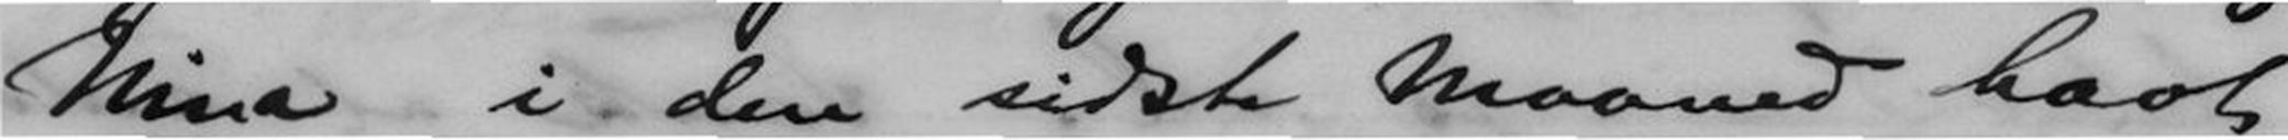Nina i den sidste Maaned havt
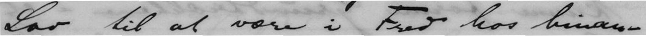Lov til at være i Fred hos hinan-
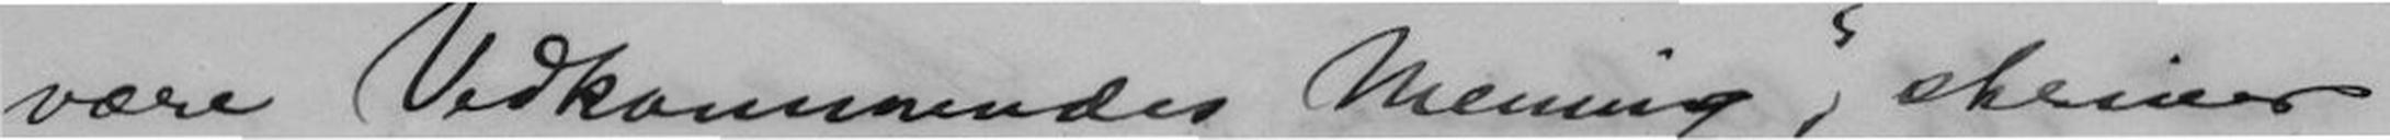være Vedkommendes Mening", skriver
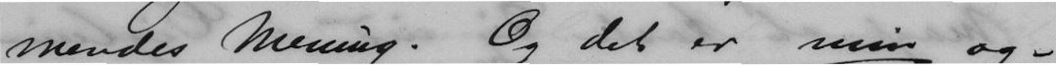mendes Mening. Og det er min og-
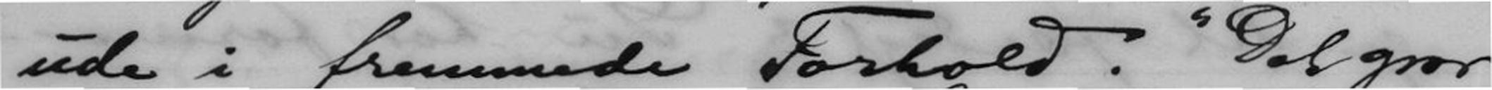ude i fremmede Forhold. "Det gror
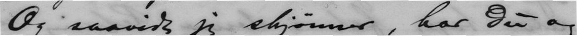Og saavidt jeg skjønner, har Du og
